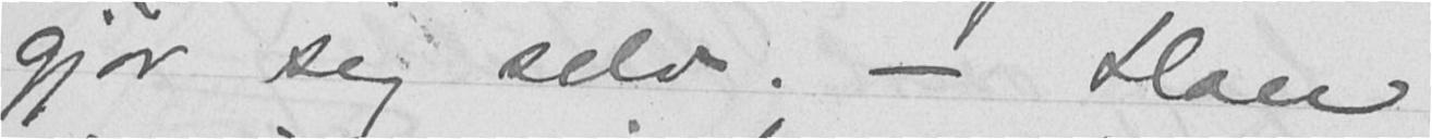gjør sig selv. - Han
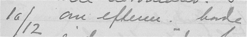16/12 Om efterm. havde
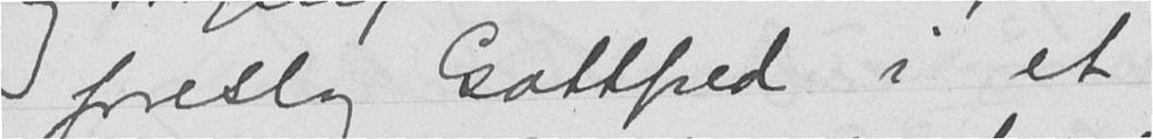foreslog Gottfred i et
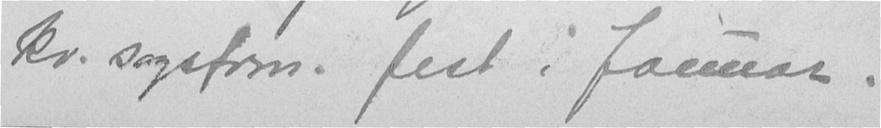kv.sagsforn. fest i Januar.
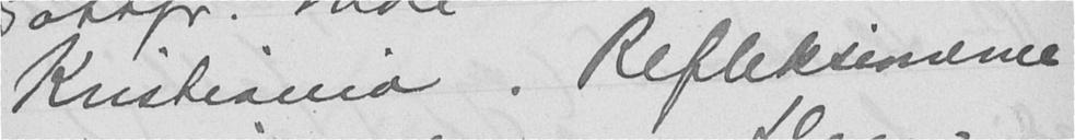Kristiania. Reflektionerne
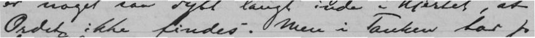Ordet ikke findes. Men i Tanken tar jeg
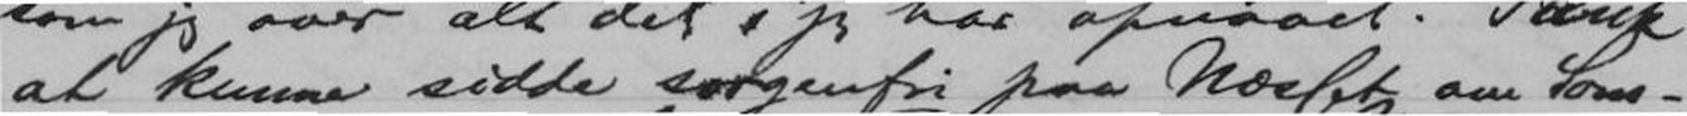at kunne sidde sorgenfri paa Næsset om Som-
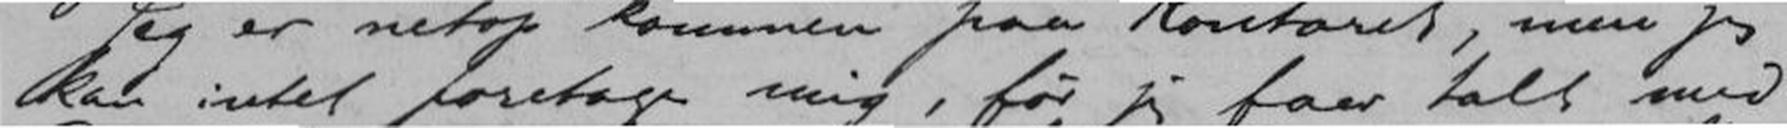kan intet foretage mig, før jeg faar talt med
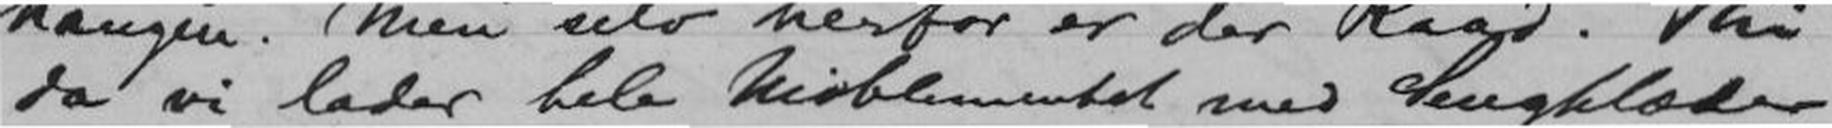da vi lader hele Møblementet med Sengeklæder
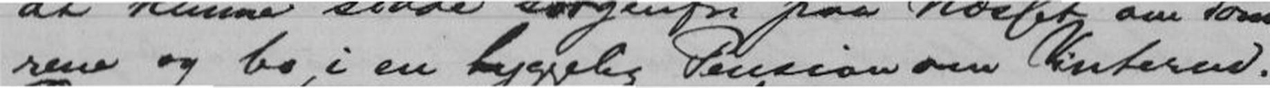rene og bo i en hyggelig Pension om Vinteren.
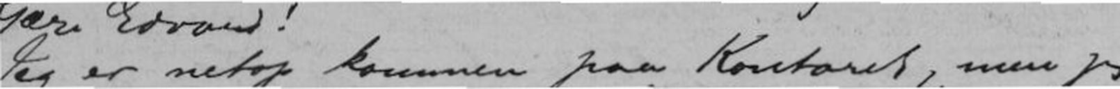Jeg er netop kommen paa Kontoret, men jeg
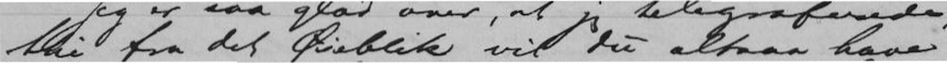thi fra det Øieblik vil du altsaa have
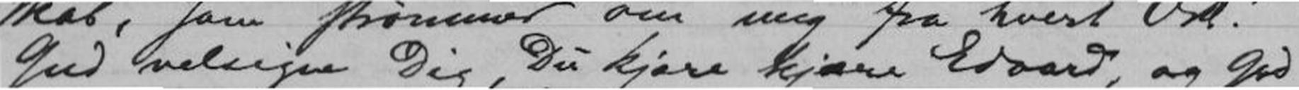Gud velsigne Dig, Du kjære kjære Edvard, og Gud
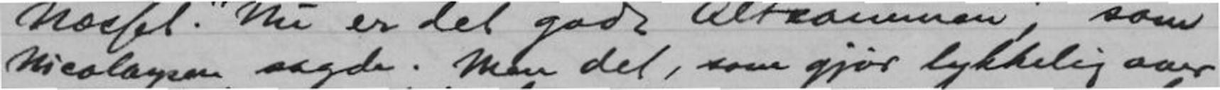Nicolaysen sagde. Men det, som gjør lykkelig over
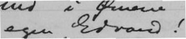egen Edvard!
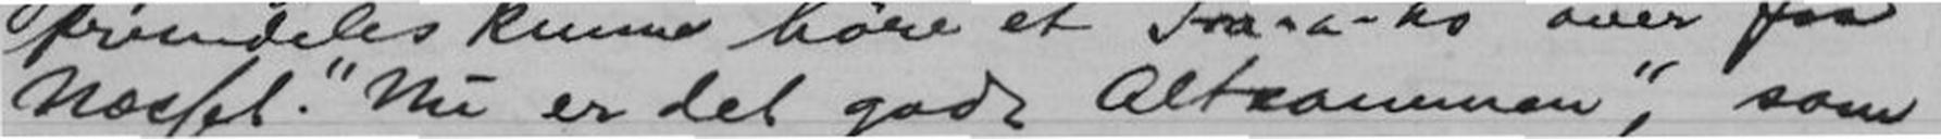Næsset. "Nu er det godt altsammen", som
